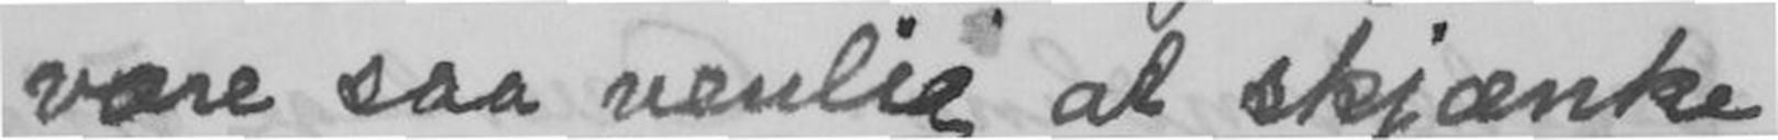være saa venlig at skjænke
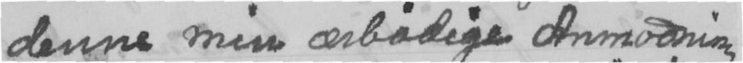denne min ærbødige Anmodning
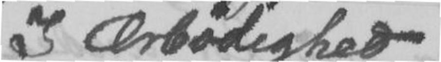I Ærbødighed
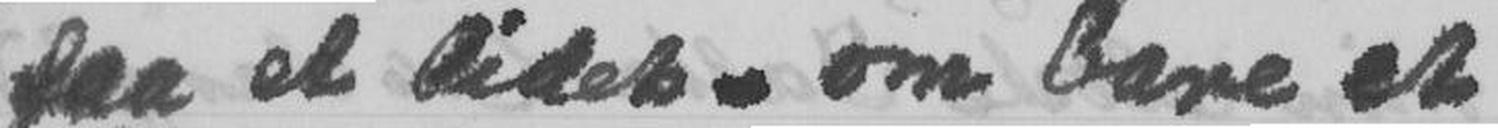faa et lidet - om bare et
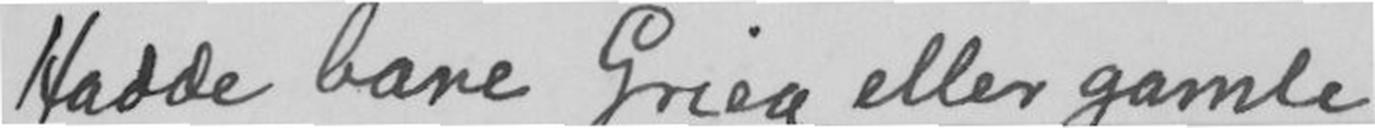Hadde bare Grieg eller gamle
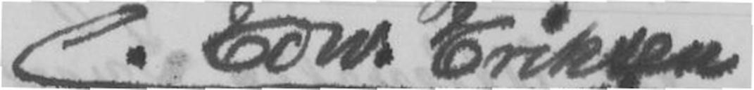Edw. Eriksen
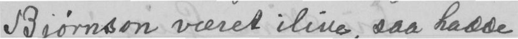Bjørnson været ilive, saa hadde
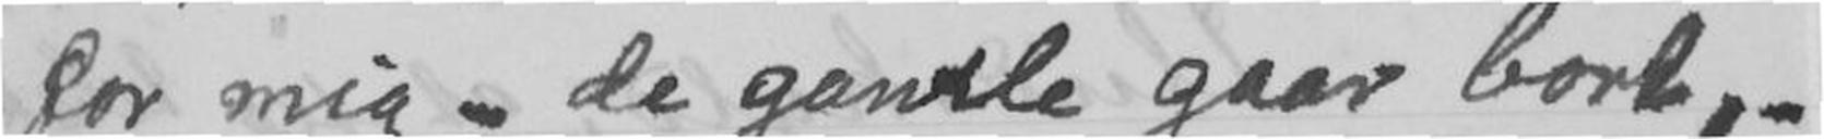for mig - de gamle gaar bort, -
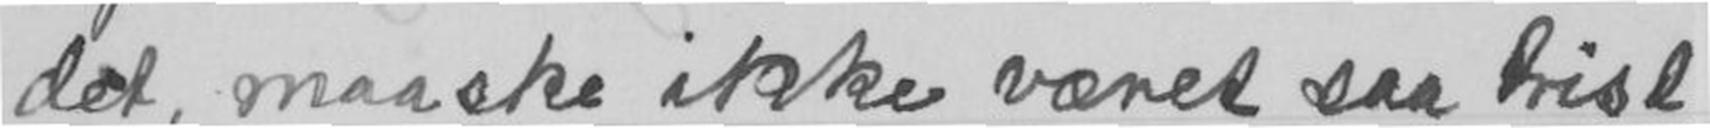det, maaske ikke været saa trist
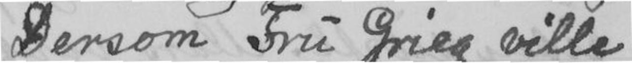Dersom Fru Grieg ville
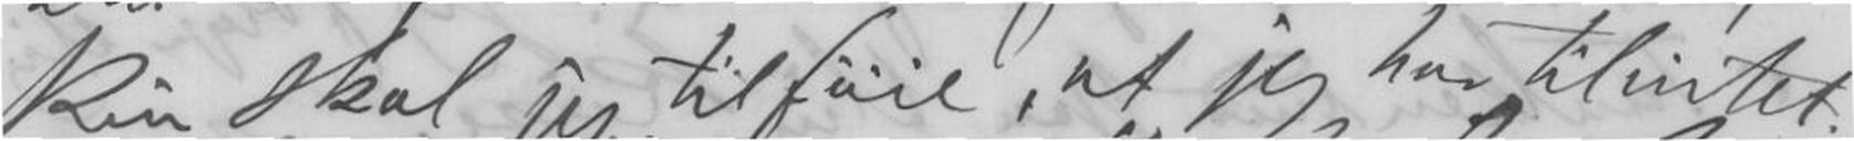kun skal jeg tilføie, at jeg har tilintet-
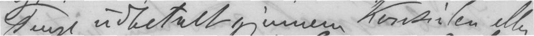Penge udbetalt gjennem Konsulen eller
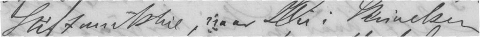Stiftamtstue, naar Du i Skrivelsen
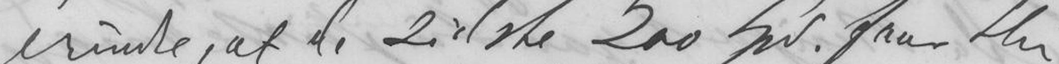erindre, at de sidste 200 Spd. faar Du
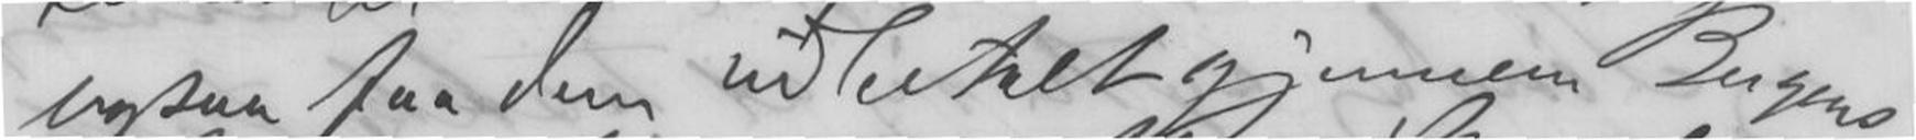ogsaa faa dem udbetalt gjennem Bergens
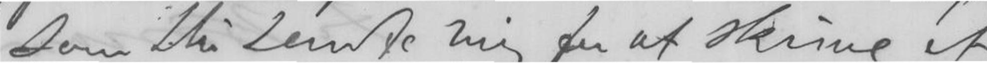som Du sendte mig for at skrive et
